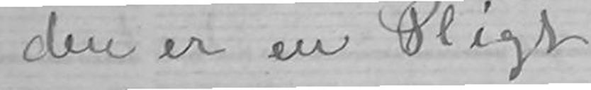den er en Pligt.
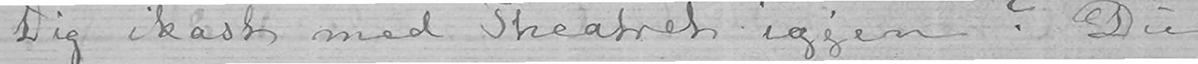Dig ikast med Theatret igjen? Du
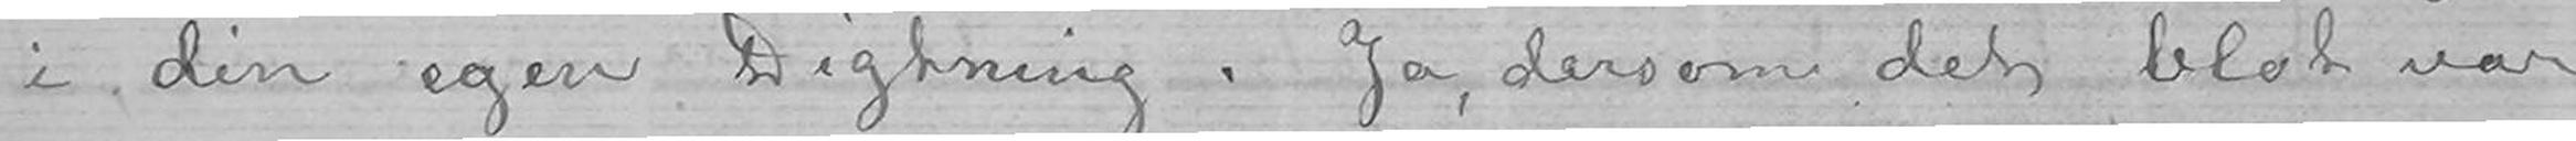i din egen Digtning. Ja, dersom det blot var
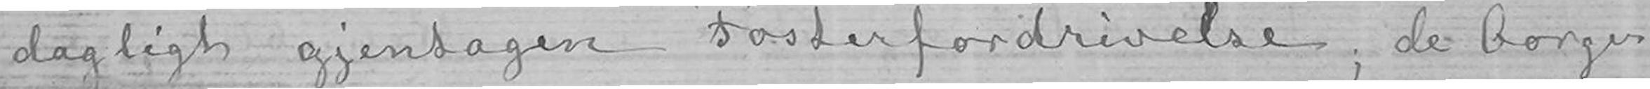dagligt gjentagen Fosterfordrivelse; de borger-
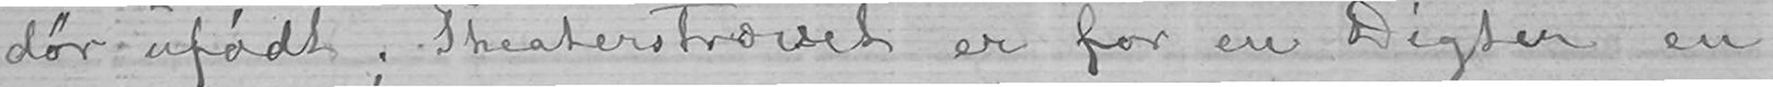dør ufødt; Theaterstrævet er for en Digter en
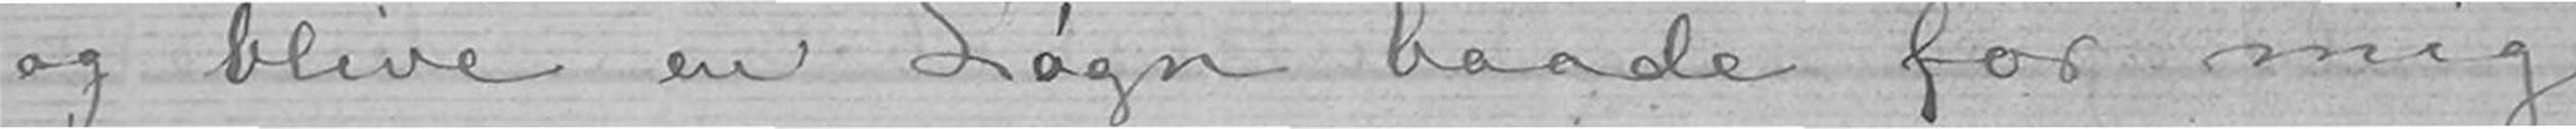og blive en Løgn baade for mig
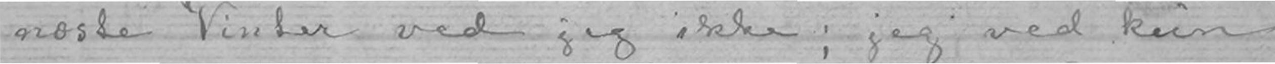næste Vinter ved jeg ikke; jeg ved kun
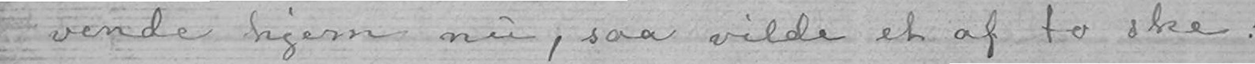vende hjem nu, saa vilde et af to ske:
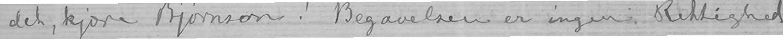det, kjære Bjørnson! Begavelsen er ingen Rettighed,
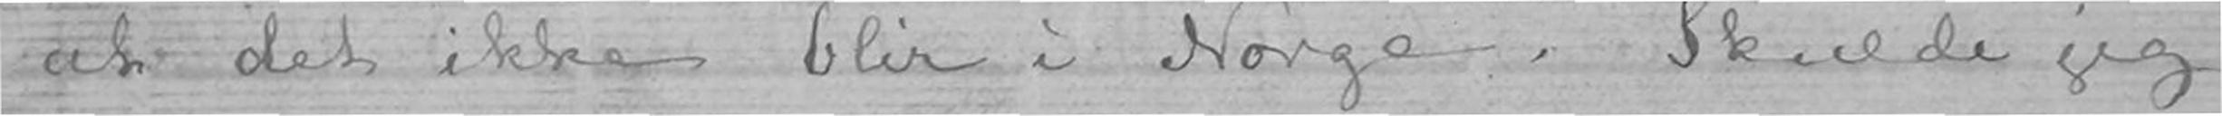at det ikke blir i Norge. Skulde jeg
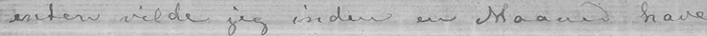enten vilde jeg inden en Maaned have
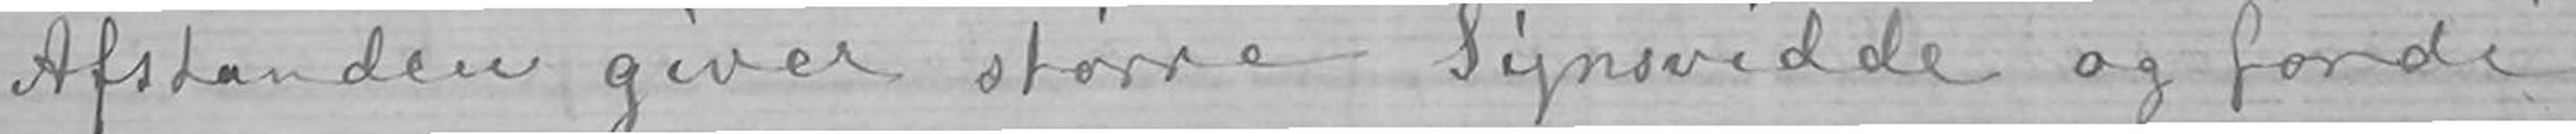Afstanden giver større Synsvidde og fordi
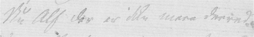Nu Alf der er ikke mere derved.
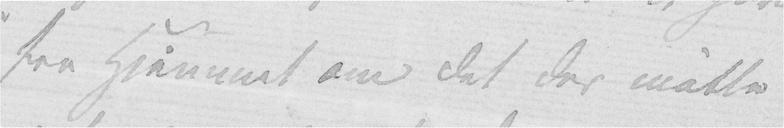fra Hjemmet om det der måtte
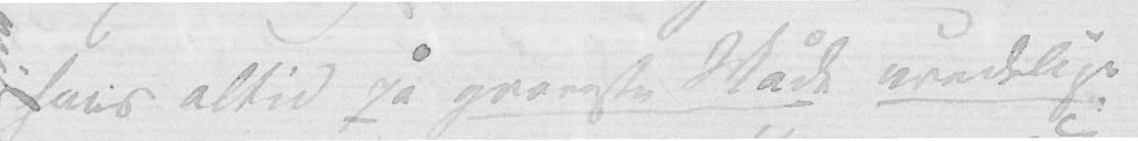hais altid på groveste Måde uredelige
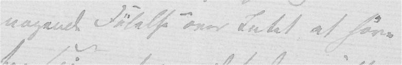nagende Følelse over Intet at høre
